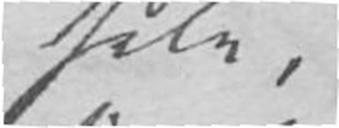selv,
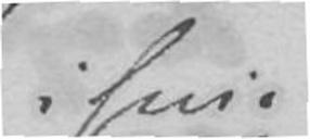i hvis
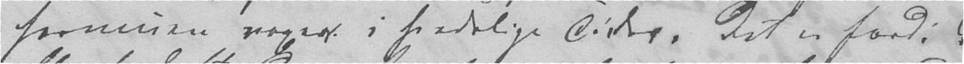formuen voxer i fredelige Tider. Det er fordi
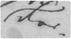For-
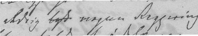aldrig nogen Regjering
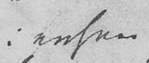i enhver
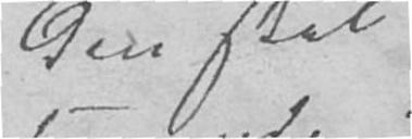den skal
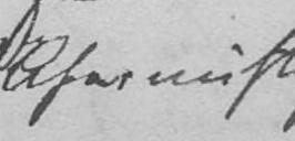Ufornuft
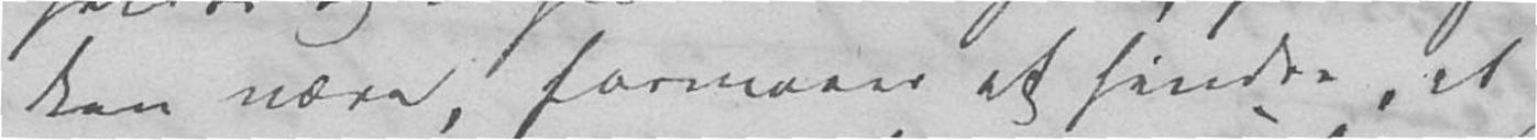kan være, formaaer at hindre, at
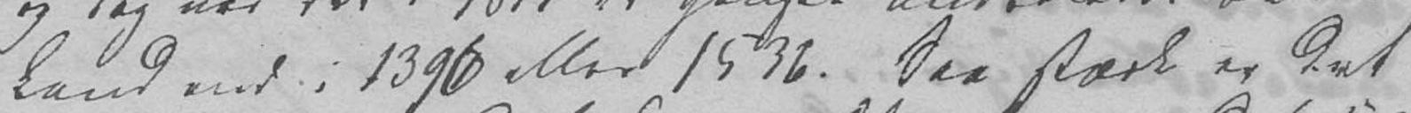Land end i 1390 eller 1536. Saa stærk er det
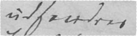udsondres
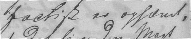factisk er ophævet,
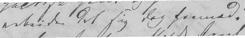arbeider det sig dog fremad.
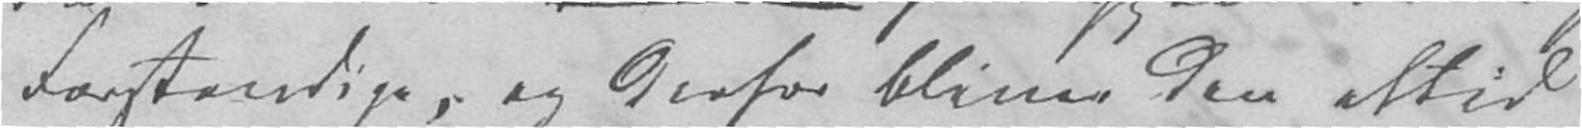Forstandige, og derfor bliver den altid
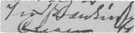Indskrænkning
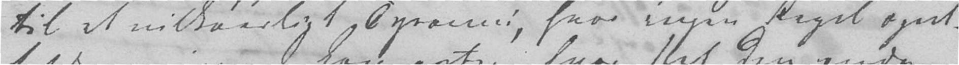til et vilkaarligt Tyranni, hvor ingen Regel opret-
